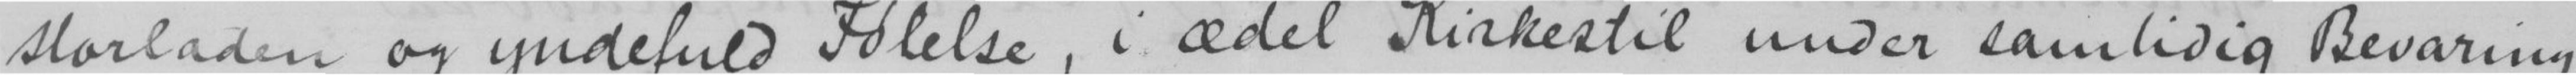storladen og yndefyld Følelse, i ædel Kirkestil under samtidig Bevaring
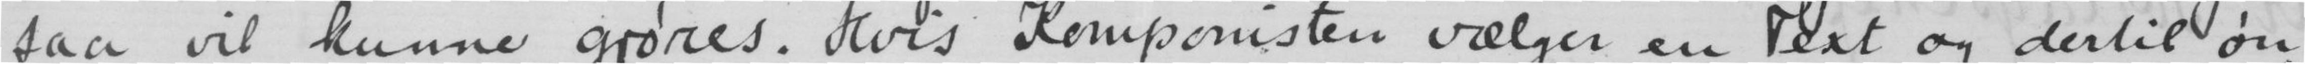saa vil kunne gjøres. Hvis Komponisten vælger en Text og dertil øn-
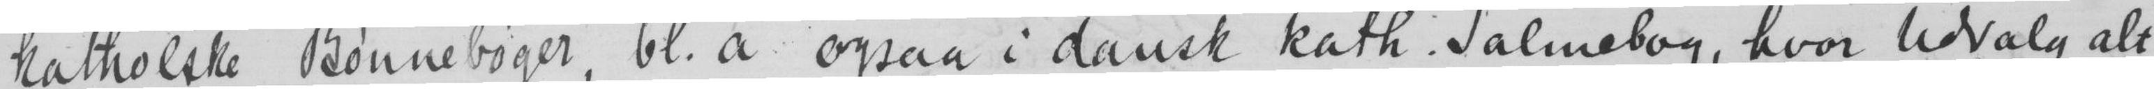katholske Bønnebøger, bl.a. ogsaa i dansk kath. Salmebog, hvor Udvalg alt
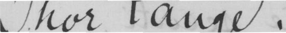Thor Lange.
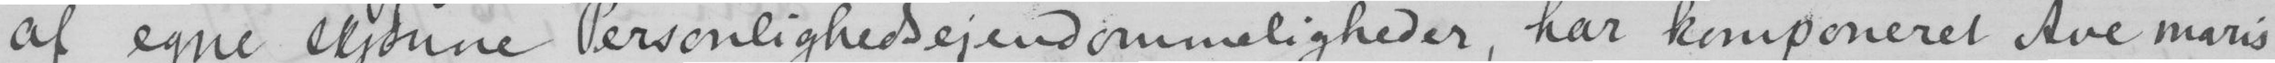af egne skjønne Personlighedsejedommeligheder, har komponeret Ave maris
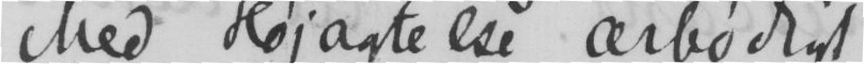Med Høiagtelse ærbødigst
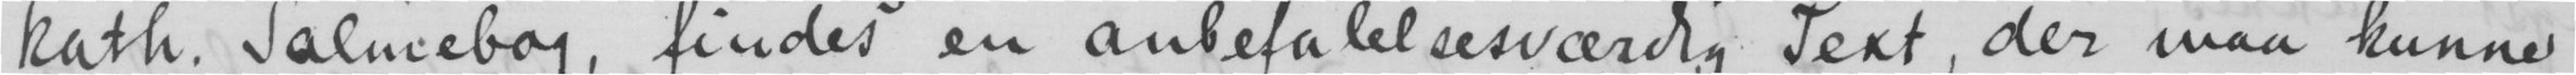kath. Salmebog, findes en anbefalelsesværdig Text, der maa kunneopsøges.
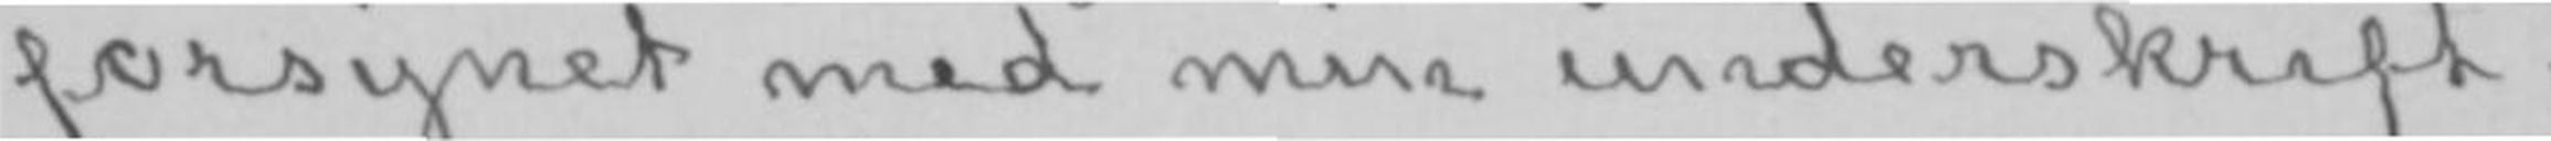forsynet med min underskrift.
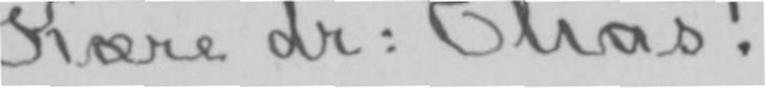Kære dr: Elias!
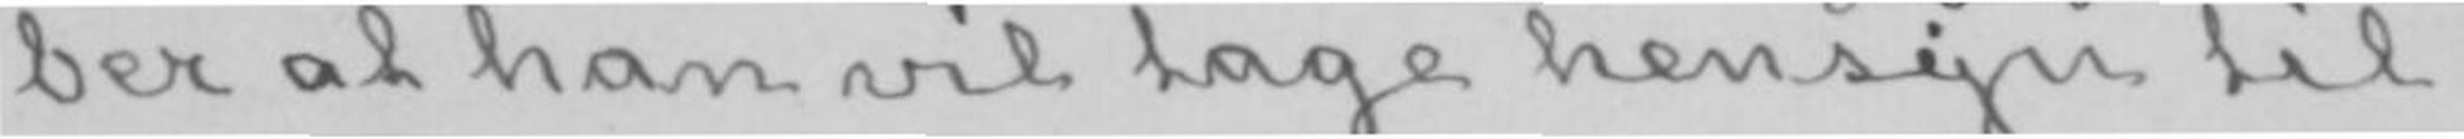ber at han vil tage hensyn til
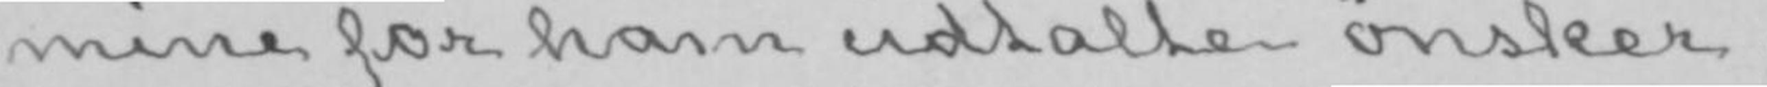mine for ham udtalte ønsker.
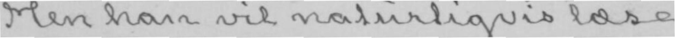Men han vil naturligvis læse
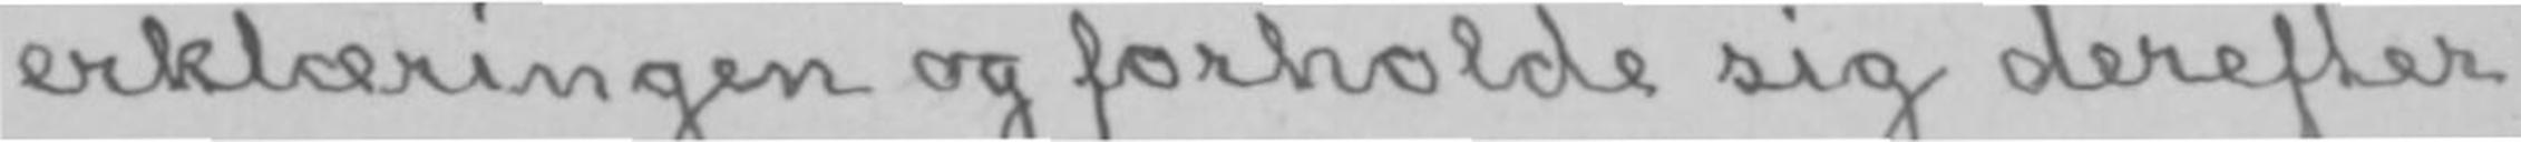erklæringen og forholde sig derefter.
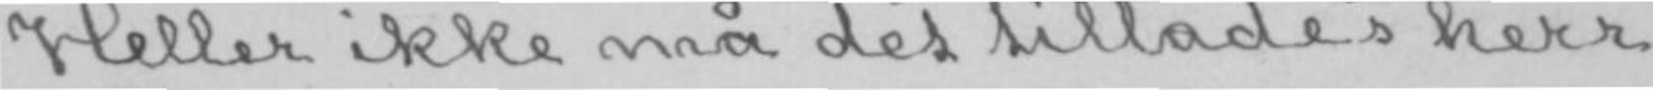Heller ikke må det tillades herr
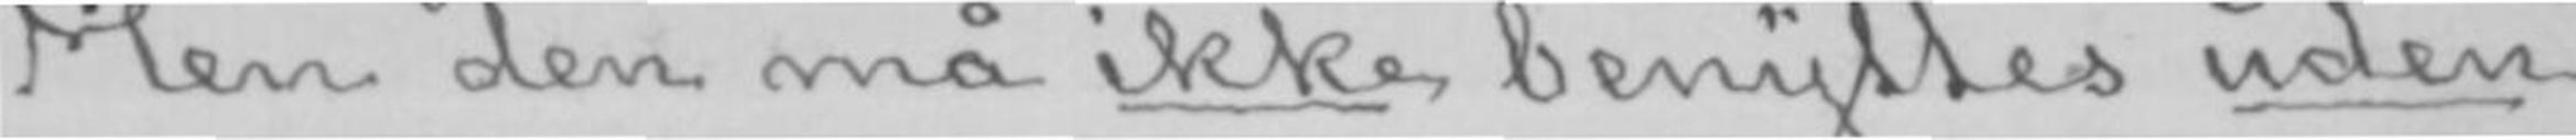Men den må ikke benyttes uden
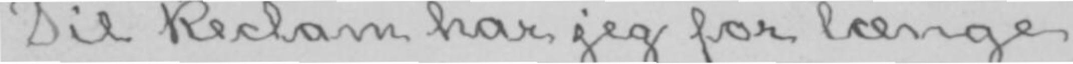Til Reclam har jeg for længe
